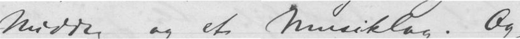Middag og et Musiklag. Og
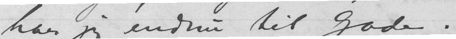har jeg endnu til Gode.
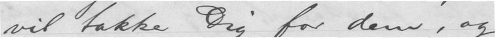vil takke Dig for dem, og
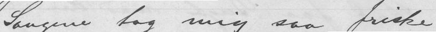Sangene tog mig saa friske
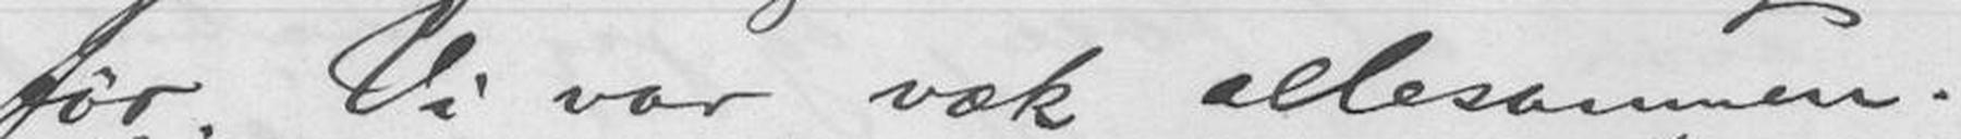før. Vi var væk allesammen.
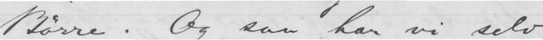Børre. Og saa har vi selv
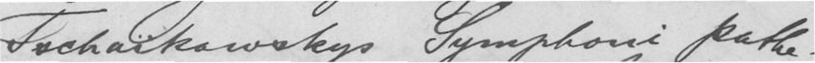Tschaikowskys Symphoni pathe-
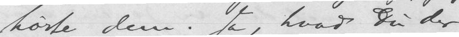hørte dem. Ja, hvad Du der
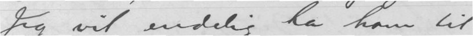Jeg vil endelig ha ham til
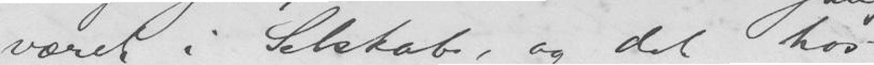været i Selskab, og det hos
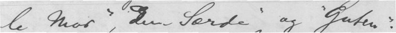le Mor", "Den Særde" og "Guten".
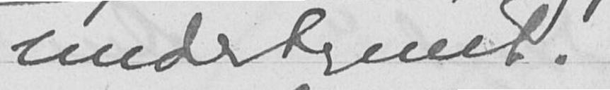undertegnet.
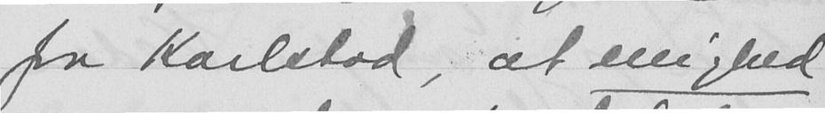fra Karlstad, at enighed
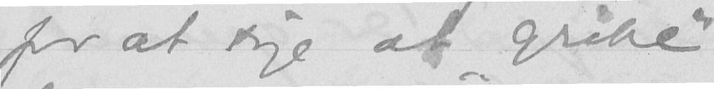for at søge at "gribe"
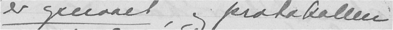er opnaaet, og protokollen
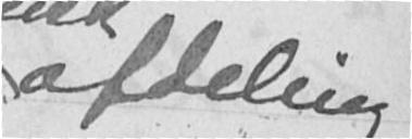afdeling
